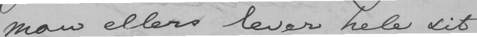man ellers lever hele sit
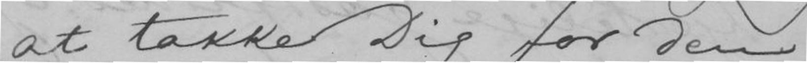at takke Dig for den
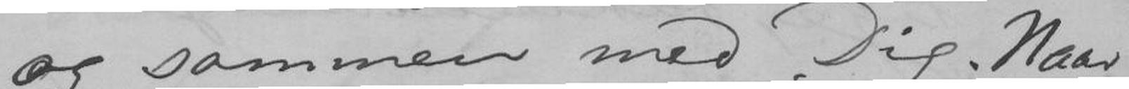og sammen med Dig. Naar
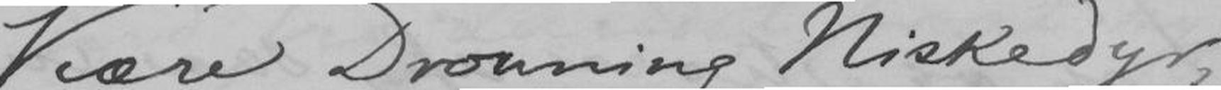Kære Dronning Niskedyr,
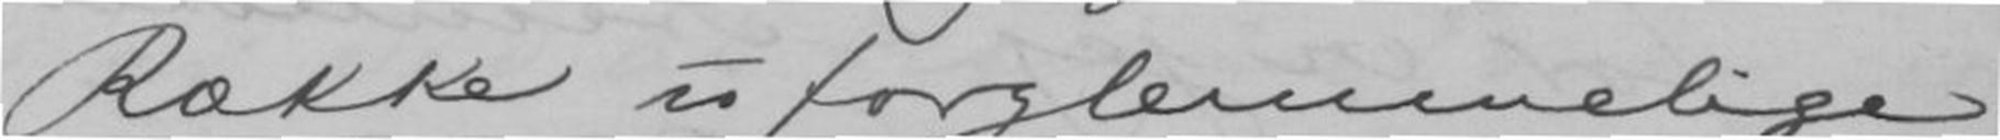Række uforglemmelige
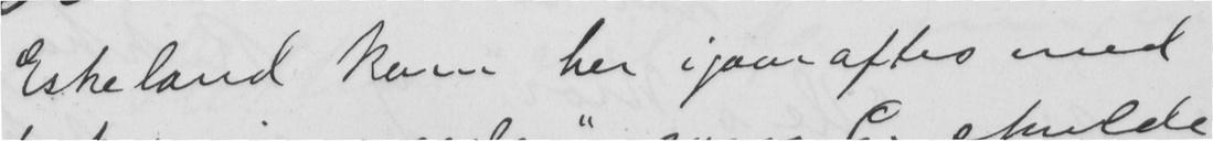Eskeland kom her igaar aftes med
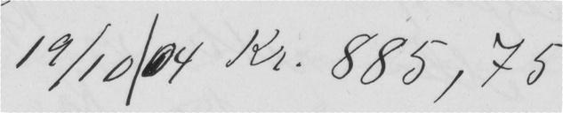19/10/04 Kr. 885,75
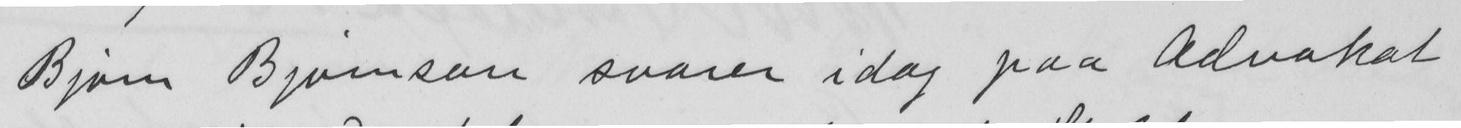Bjørn Bjørnson svarer idag paa Advokat
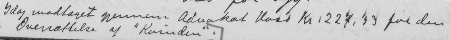Idag modtaget gjennem Advokat Voss Kr. 227,33 for den
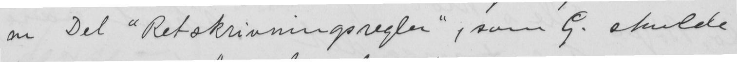en Del "Retskrivningsregler", som G. skulde
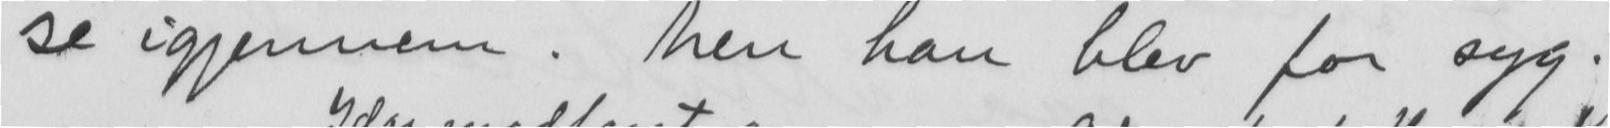se igjennem. Men han blev for syg.
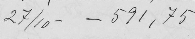27/10- - 591,75
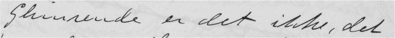Glimrende er det ikke, det
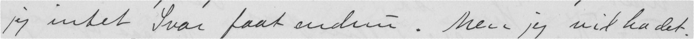jeg intet Svar faat endnu. Men jeg vil ha det.
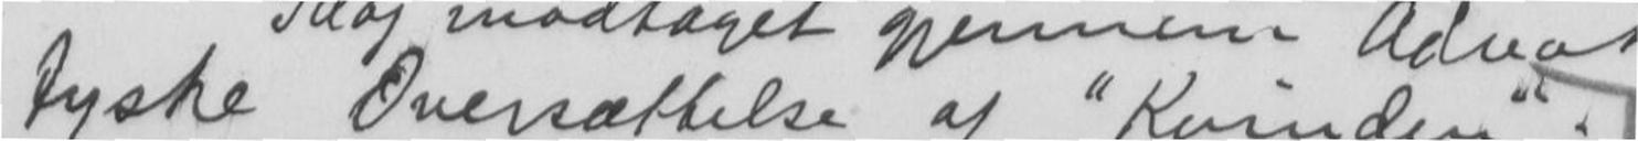tyske Oversættelse af "Kvinden".
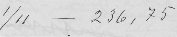1/11 -- 236,75
